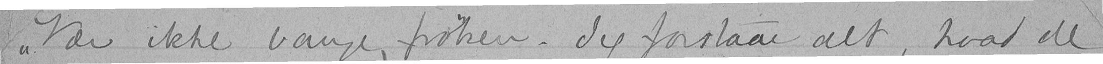"Vær ikke bange frøken. Jeg forstaar alt, hvad de
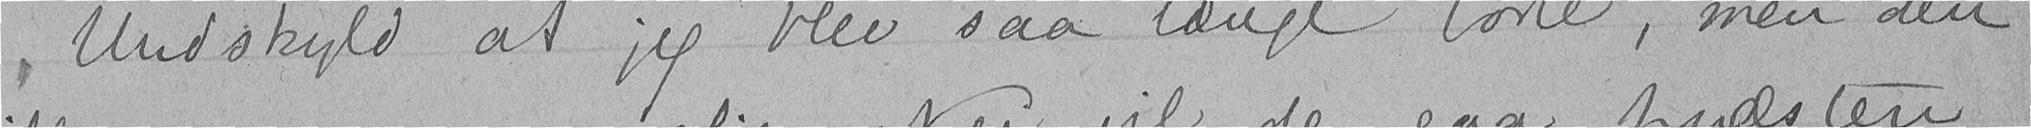"Undskyld at jeg blev saa længe borte, men den
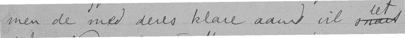men de med deres klare aand vil snart
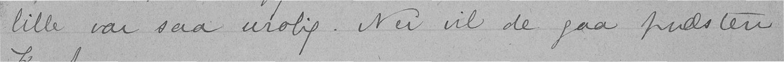lille var saa urolig. Nei vil de gaa præsten
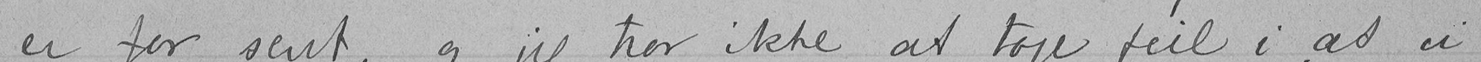er for sent, og jeg tror ikke at tage feil i, at vi
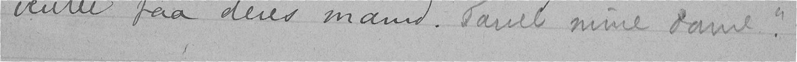venter paa deres mand. Farvel mine dame."
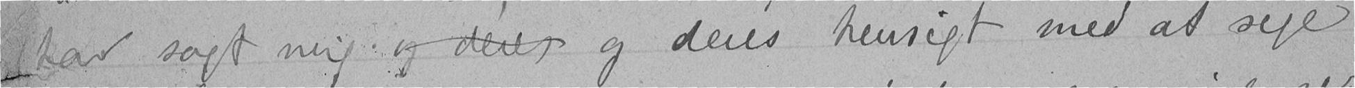har sagt mig og deres og deres hensigt med at sige
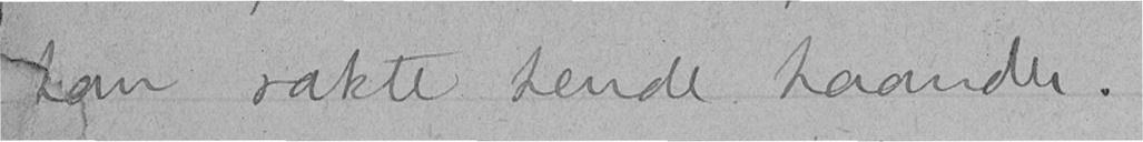han rakte hende haanden.
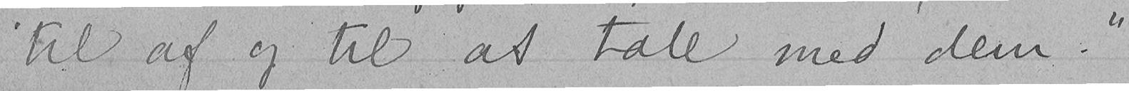til af og til at tale med dem."
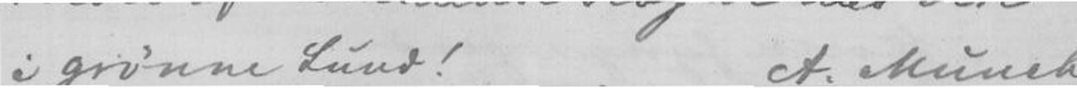i grønne Lund! A. Munch.
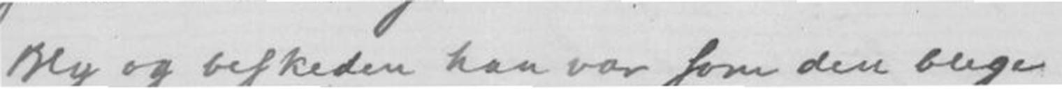Sky og beskeden han var som den blege
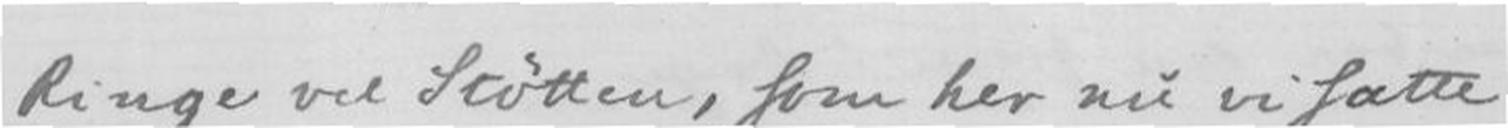Ringe ved Støtten, som her nu vi satte,
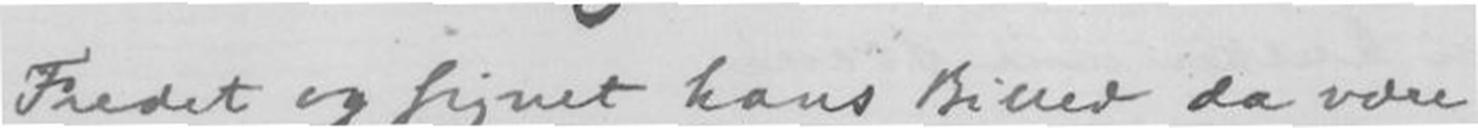Fredet og signet hans Billed da være
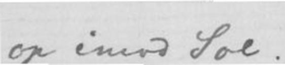op imod Sol.
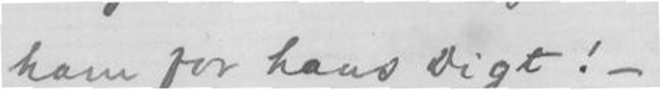ham for hans Digt! -
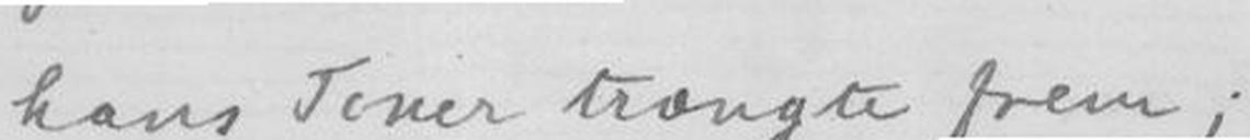hans Toner trængte frem;
